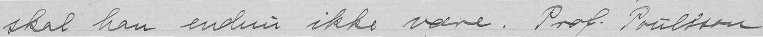skal han endnu ikke være. Prof. Poulssen
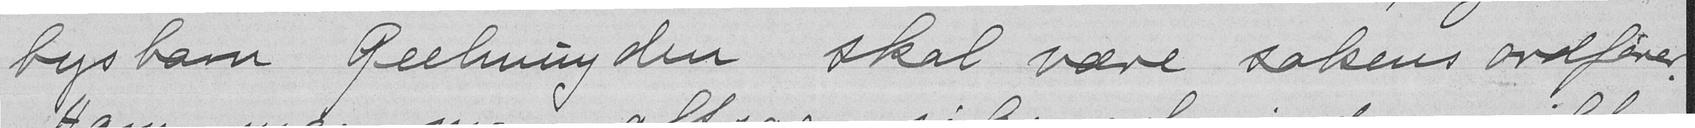bys barn Geelmuyden skal være sakens ordfører.
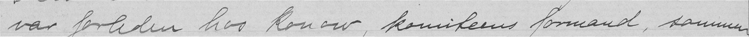var forleden hos Konow, komiteens Formand, samme
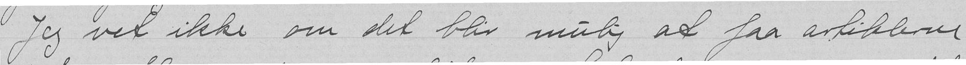Jeg ved ikke om det bliv mulig at faa artiklerne
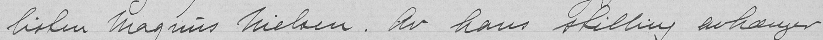listen Magnus Nielsen. Av hans stilling avhænger
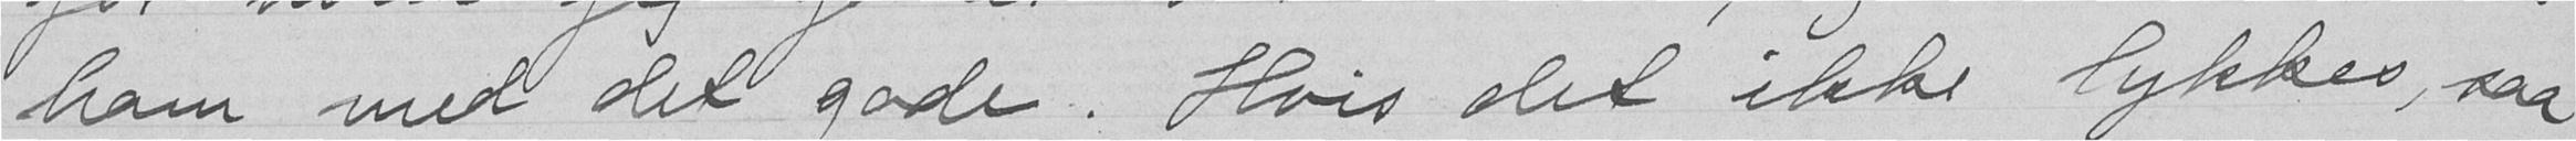ham med det gode. Hvis det ikke lykkes, saa
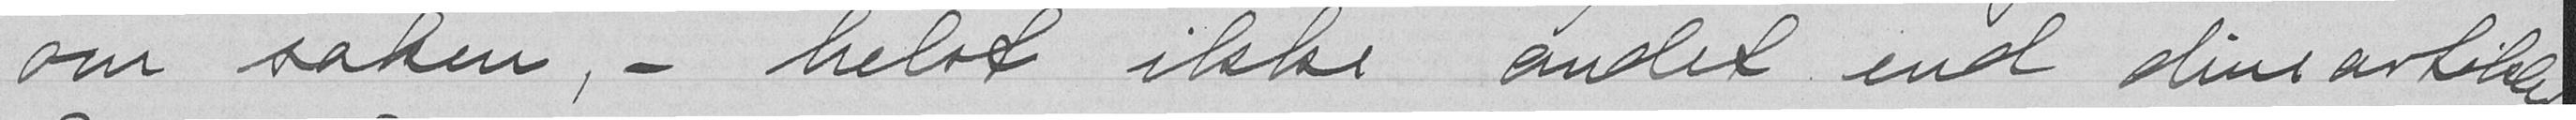om sake, - helst ikke andet end dine artikler.
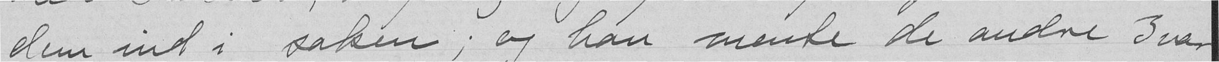dlem ind i saken; og han mente de andre 3 var
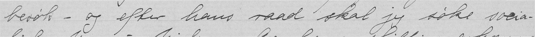besøk - og efter hans raad skal jeg søke socia-
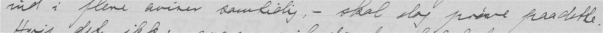ind i flere aviser samtidlig, - skal dog prøve paadette.
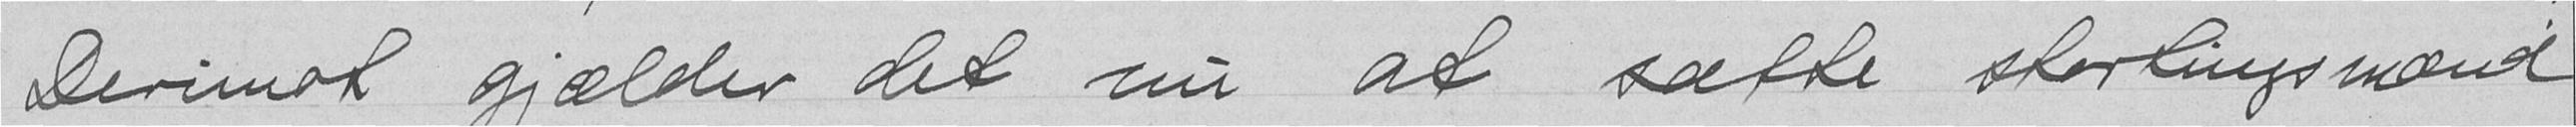Derimod gjælder det nu at sætte stortingsmænd
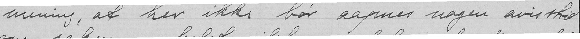mening, at her ikke bør aapnes nogen avisstrid
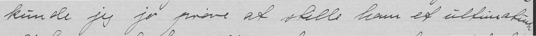kunde jeg jo prøve at stille ham et ultimatum
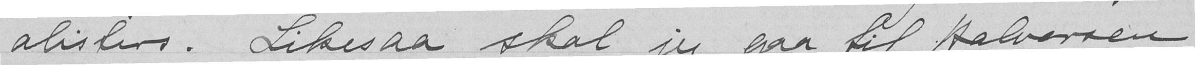alisters. Likesaa skal jeg gaa til Halvorsen
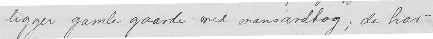ligger gamle gaarde med mansardtag; de har
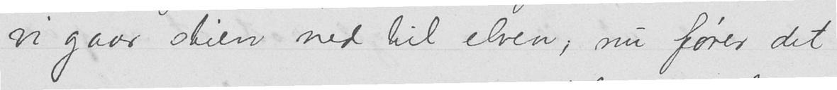vi gaar stien ned til elven, nu fører det
